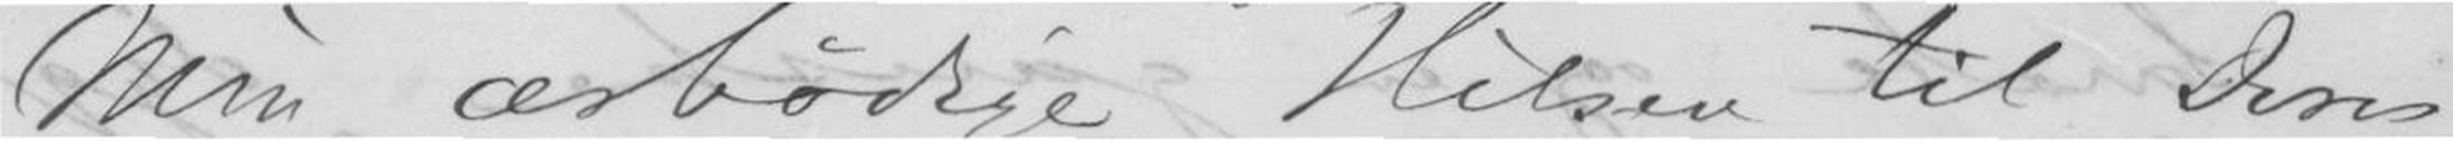Min ærbødige Hilsen til Deres
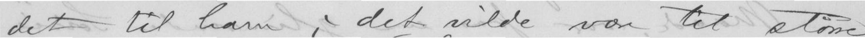det til ham; det vilde være til større
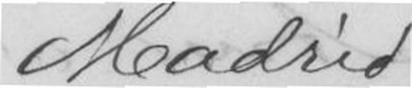Madrid
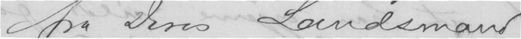fra Deres Landsmand
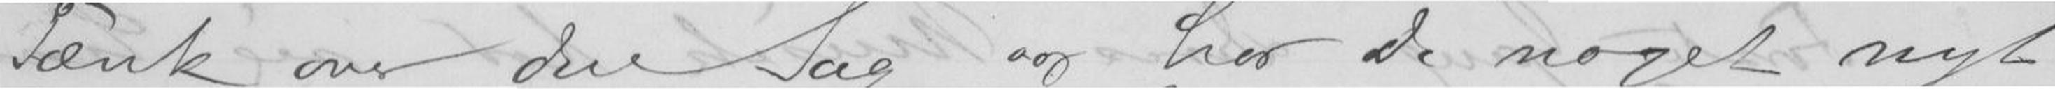Tænk over den Sag og har De noget nyt
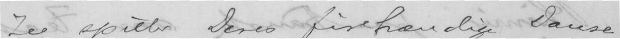Jeg spiller Deres Firehændige Danse
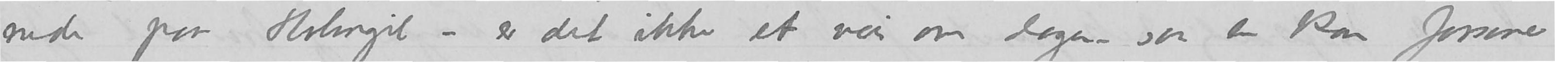nede paa Holmgil – er det ikke et veir om dagen saa en kan forsone
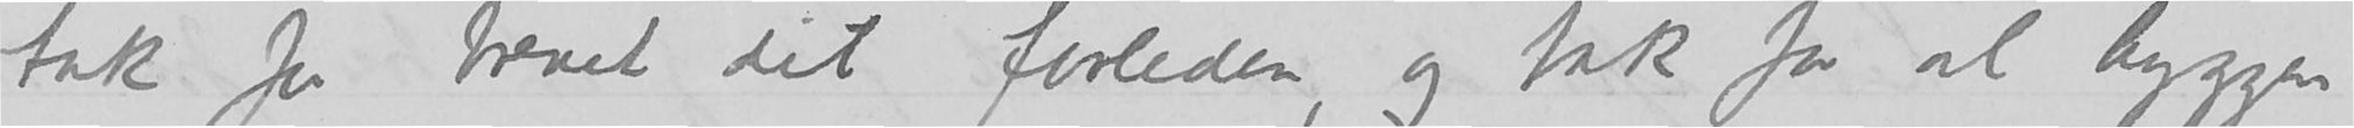Tak for brevet dit forleden, og tak for al hyggen
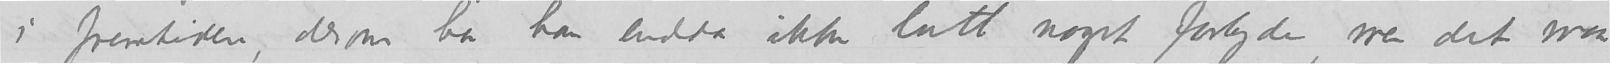i fremtiden, derom har han endda ikke latt noget forlyde, men det som maa
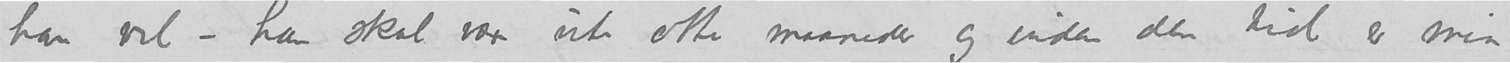han vil vel – han skal være ute otte maaneder og under den tid er min
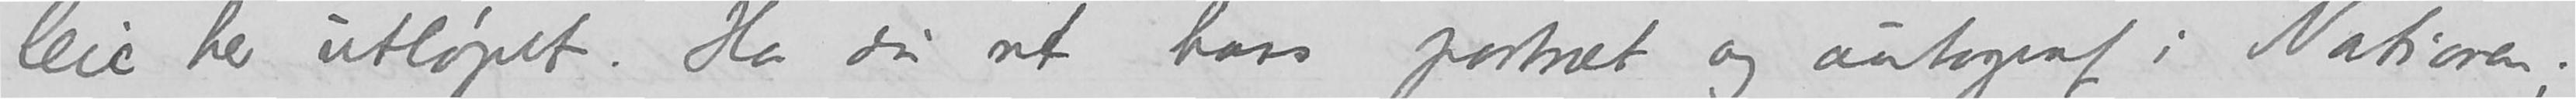leie her utløpet. Har du set hans portræt og autograf i Nationen;
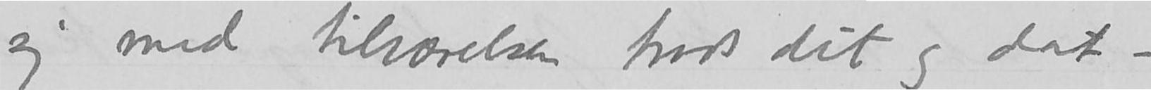sig med tilværelsen trodss dit og dat -
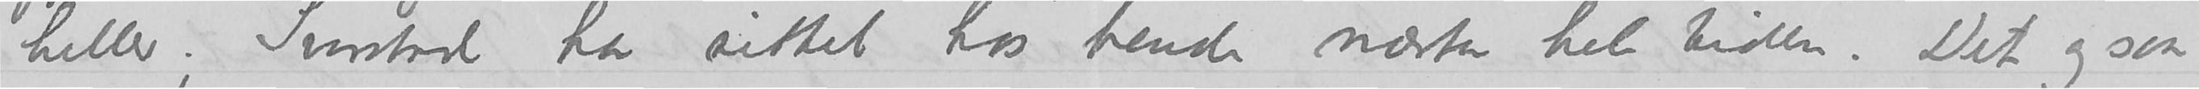heller; Svarstad har sittet hos hende næsten hele tiden. Det og saa
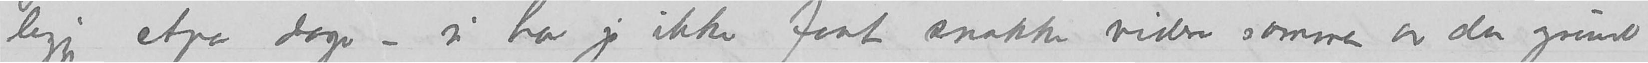ligge etpar dager – vi har jo ikke faat snakke videre sammen av den grund heller; Svarstad har sittet hos hende næsten hele tiden.
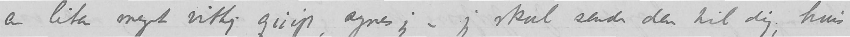en liten meget vittig quip, synes jeg - jeg skal sende den til dig, hvis
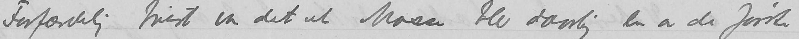Forfærdelig trist vaer det at Mosse blev daarlig en av de første
